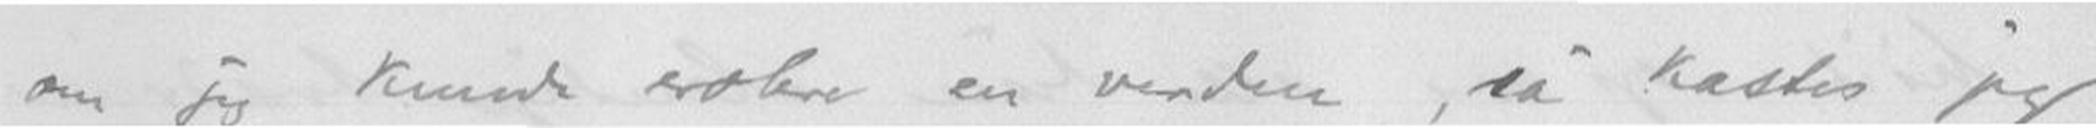om jeg kunde erobre en verden, så kastes jeg
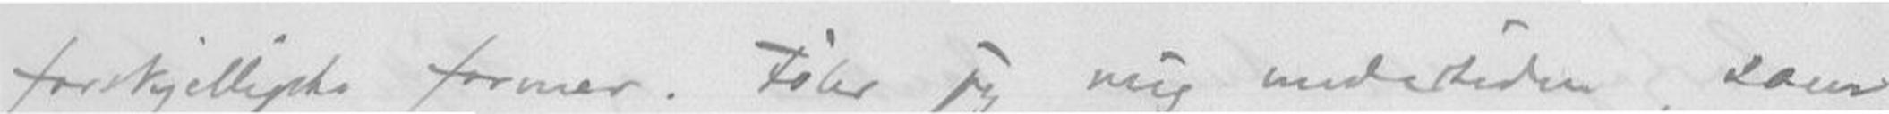forskjelligste former. Føler jeg mig undertiden, som
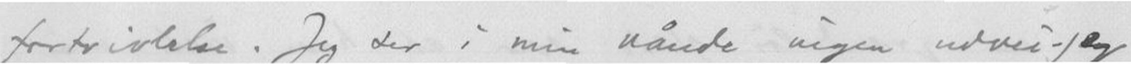fortvivlelse. Jeg ser i min vånde ingen udvei, - jeg
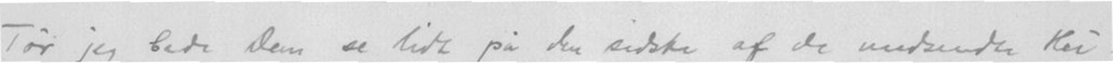Tør jeg bede Dem se lidt på den sidste af de indsendte Kei-
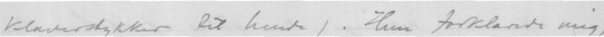klaverstykker til hende). Hun forklarede mig,
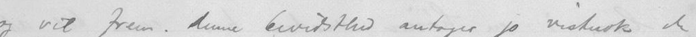og vil frem. Denne bevidshed antager jo vistnok de
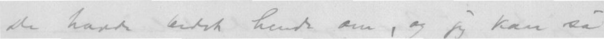De havde bedet hende om, og jeg kan så
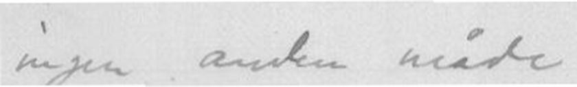ingen anden måde
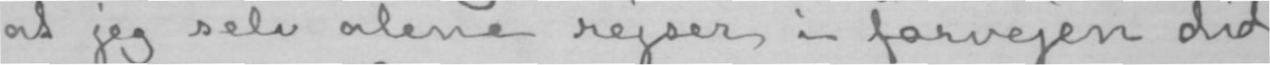at jeg selv alene rejser i forvejen did
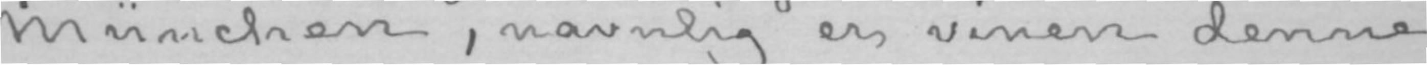München, navnlig er vinen denne
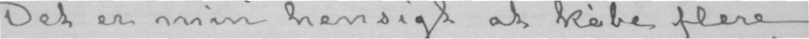Det er min hensigt at købe flere
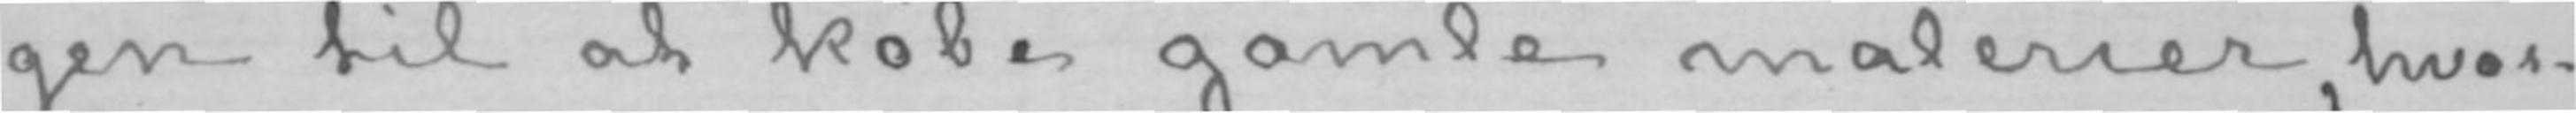gen til at købe gamle malerier, hvor-
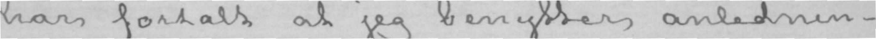har fortalt at jeg benytter anlednin-
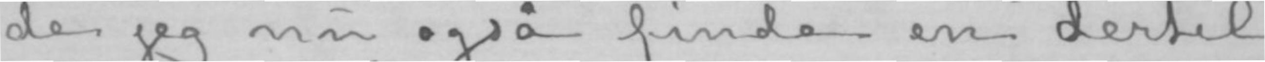de jeg nu også finde en dertil
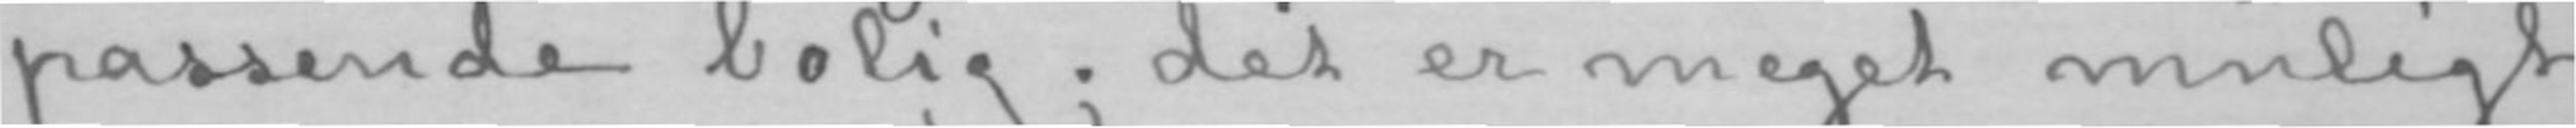passende bolig; det er meget muligt
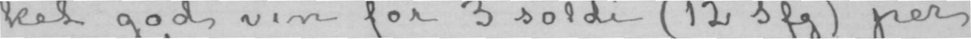ket god vin for 3 soldi (12 Pfg) per
